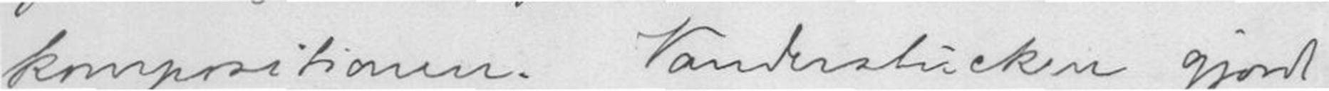kompositionen. Vanderstücken gjordt
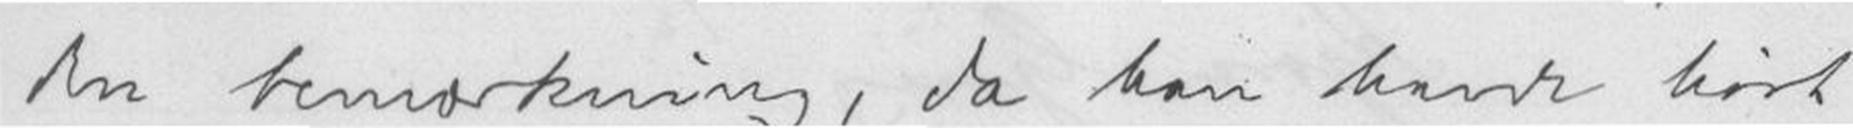den bemærkning, da han havde hørt
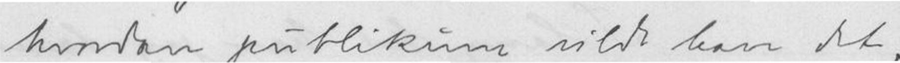hvordan publikum vilde have det,
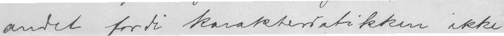andet fordi karakteristikken ikke
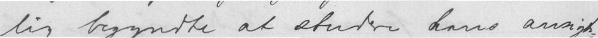lig begyndte at studere hans ansigt-
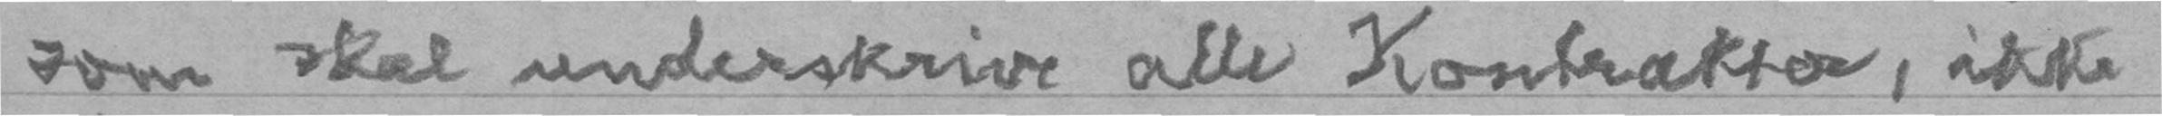som skal underskrive alle Kontrakter, ikke
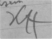KH
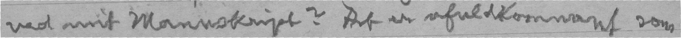ved mit Manuskript? Det er ufuldkomment som
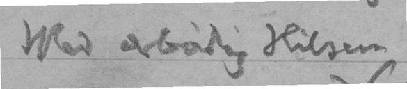Med ærbødig Hilsen
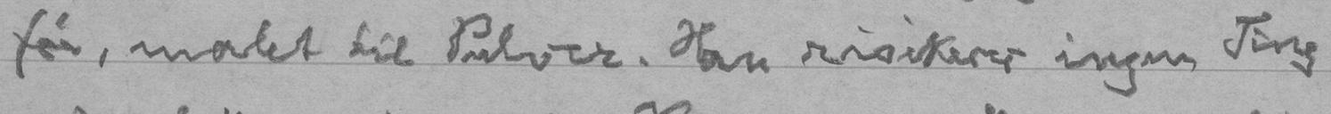før, malet til Pulver. Han risikerer ingen Ting
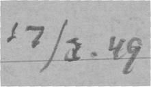17/3. 49
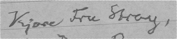Kjære Fru Stray,
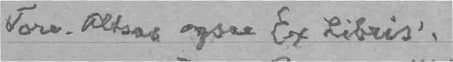Tore. Altsaa ogsaa Ex Libris'.
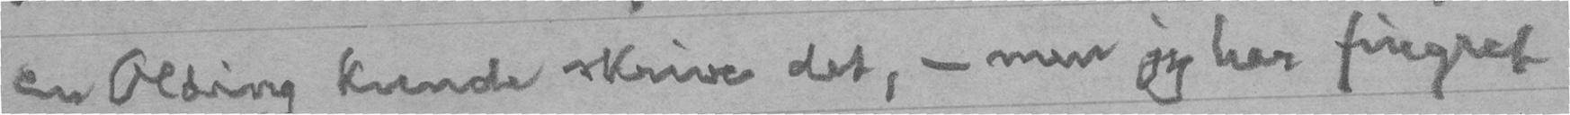en Olding kunde skrive det, - men jeg har fingret
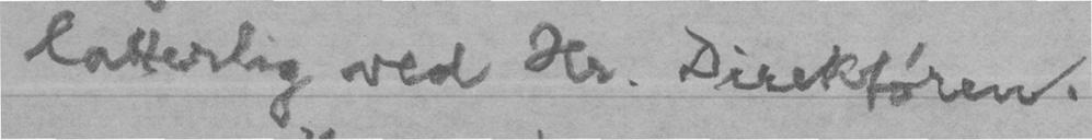latterlig ved Hr. Direktøren.
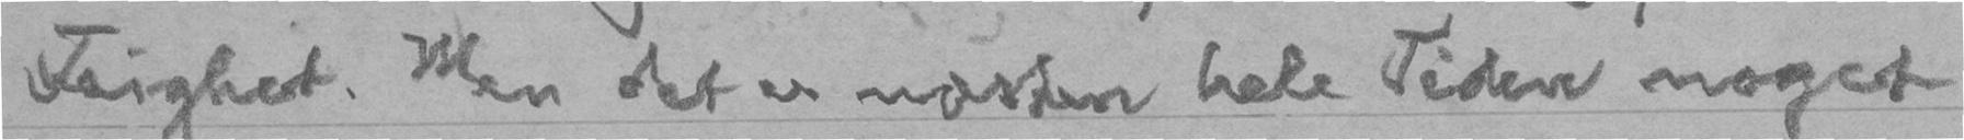Feighet. Men det er næsten hele Tiden noget
